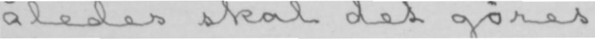Således skal det gøres!
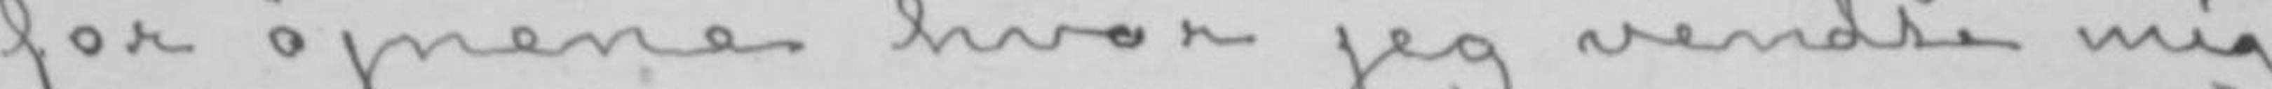for øjnene hvor jeg vendte mig,
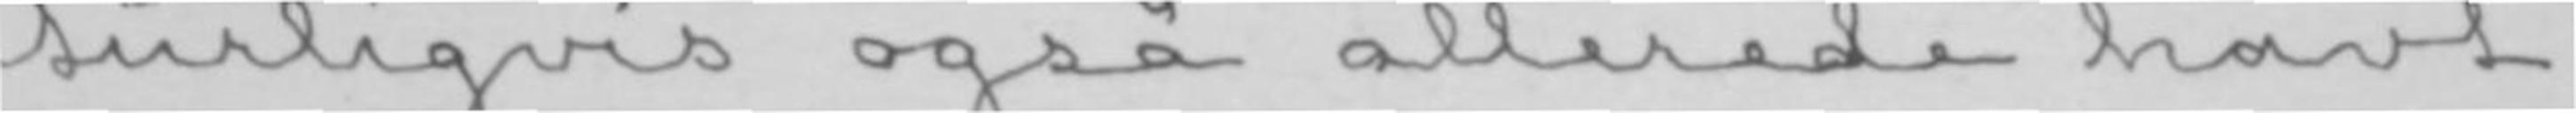turligvis også allerede havt
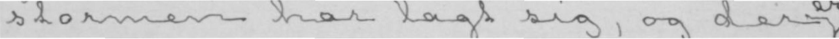stormen har lagt sig, og der er
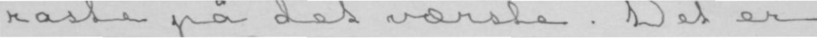raste på det værste. Det er
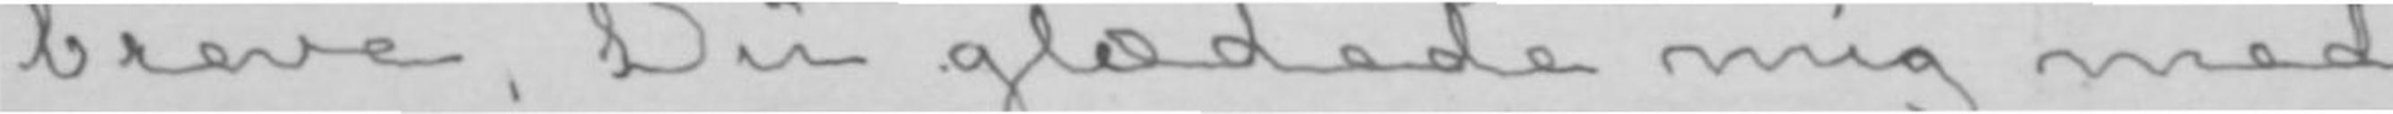breve, Du glædede mig med
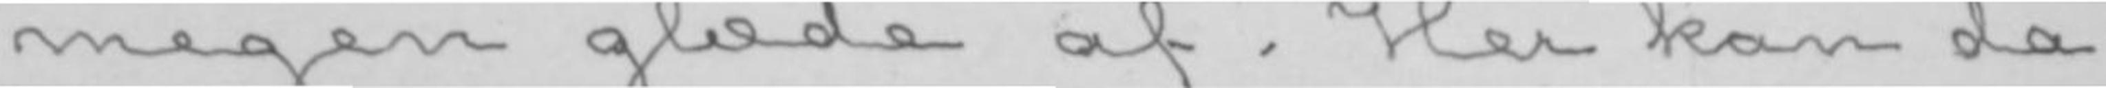megen glæde af. Her kan da
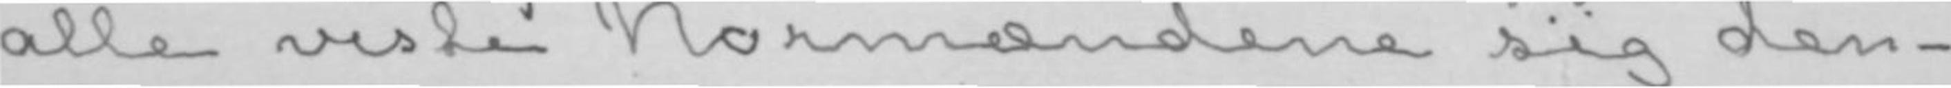alle viste Normændene sig den-
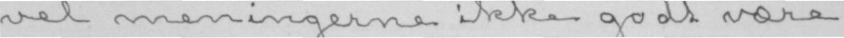vel meningerne ikke godt være
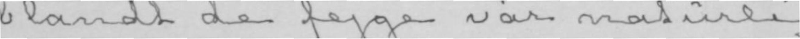blandt de fejge var naturlig-
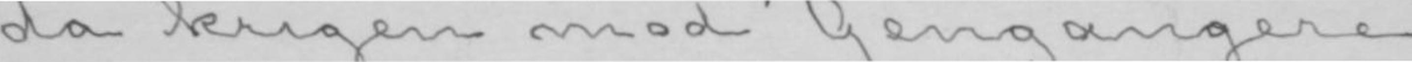da krigen mod «Gengangere»
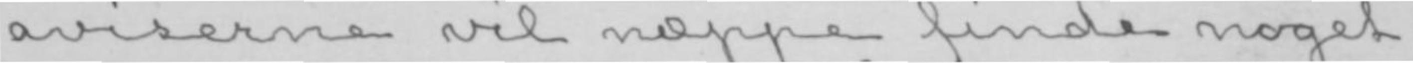aviserne vil næppe finde noget
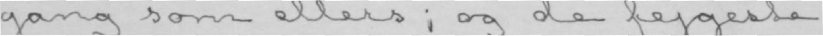gang som ellers; og de fejgeste
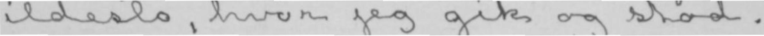sildeslo, hvor jeg gik og stod.
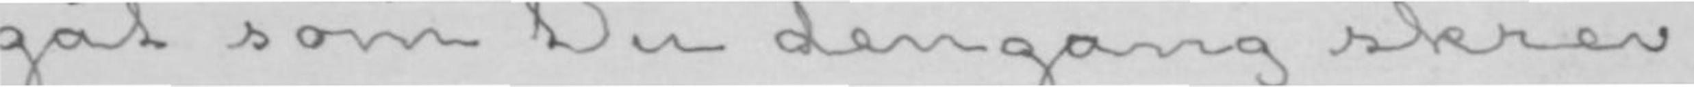gåt som Du dengang skrev;
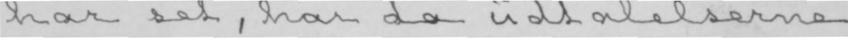har set, har da udtalelserne
